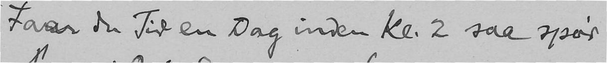Faar du Tid en Dag inden Kl. 2 saa spør
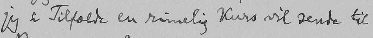jeg i Tilfælde en rimelig Kurs vil sende til
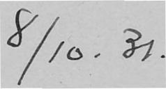8/10. 31.
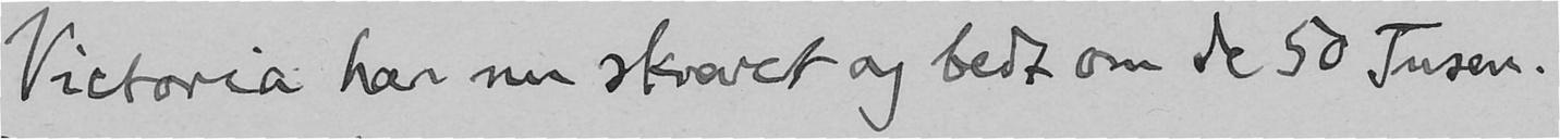Victoria har nu skrevet og bedt om de 50 Tusen.
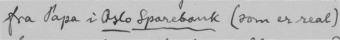fra Papa i Oslo Sparebank (som er real)
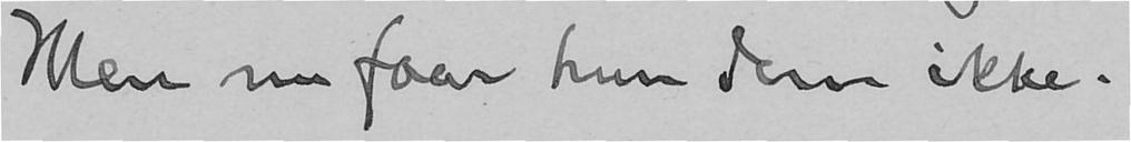Men nu faar hun dem ikke.
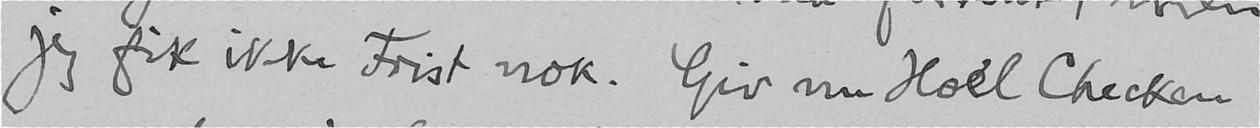jeg fik ikke Frist nok. Giv nu Hoel Checken
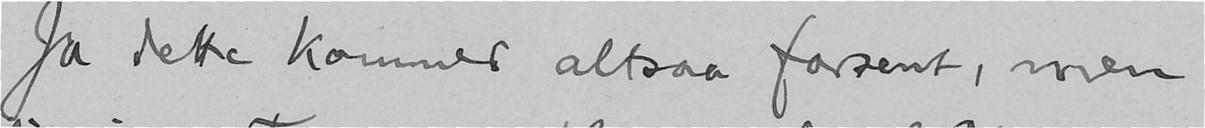Ja dette kommer altsaa forsent, men
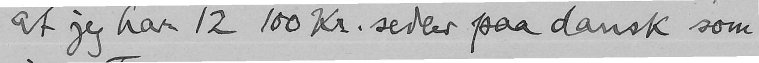at jeg har 12 100 Kr. sedler paa dansk som
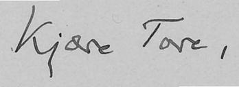Kjære Tore,
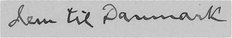dem til Danmark
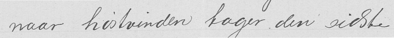naar høstvinden tager den sidste
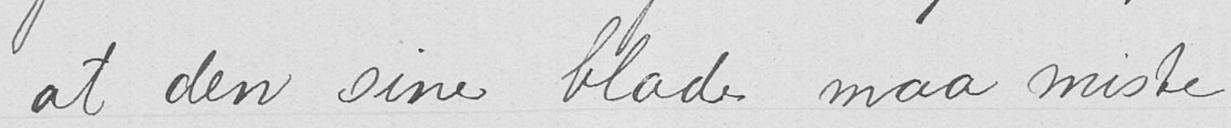at den sine blade maa miste
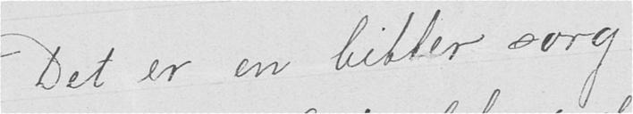Det er en bitter sorg
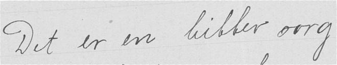Det er en bitter sorg,
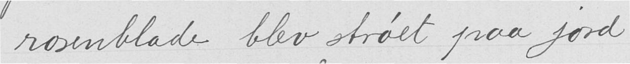rosenblade blev strøet paa jord
